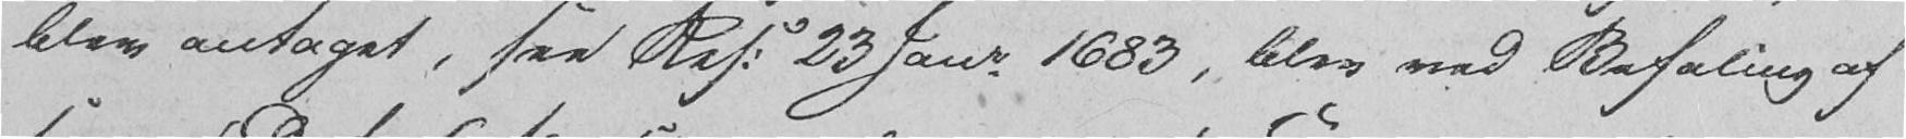blev antaget, see Res. 23 Janr. 1683, blev med Befaling af
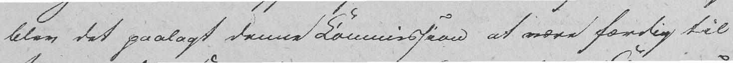blev det paalagt denne Kommission at være færdig til
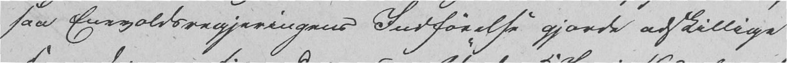saa Enevoldsregjeringens Indførelse gjorde adskillige
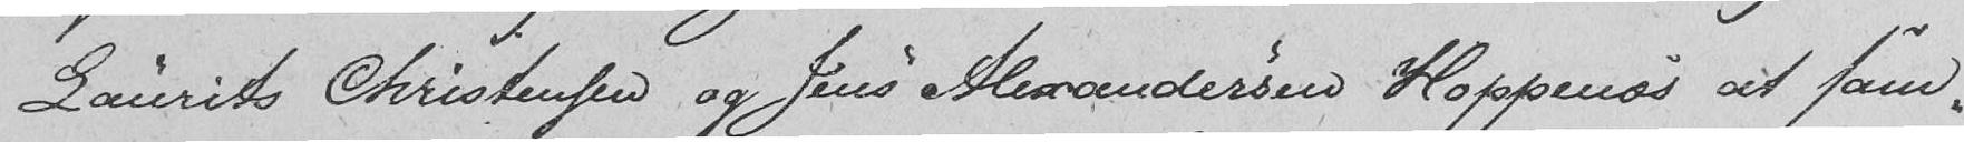Laurits Christensen og Jens Alexandersen Hoppenæs at sam-
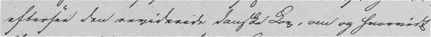eftersaa den reviderede danske Lov, om og hvorvidt
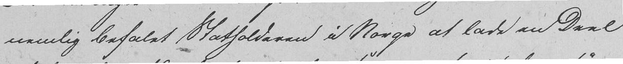nemlig befalet Statholderen i Norge at lade en Deel
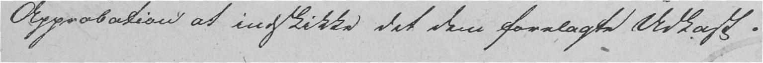Approbation at indskikke det dem forelagte Udkast.
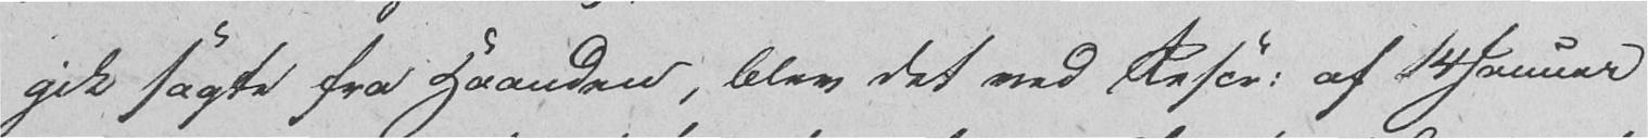gik sagte fra Haanden, blev det med Rescr. af 14 Januar
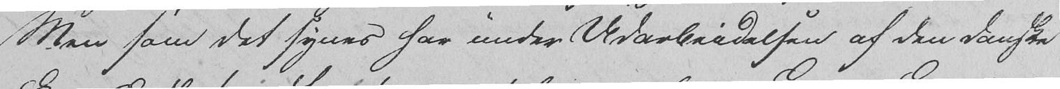Men som det synes har under Udarbeidelsen af den danske
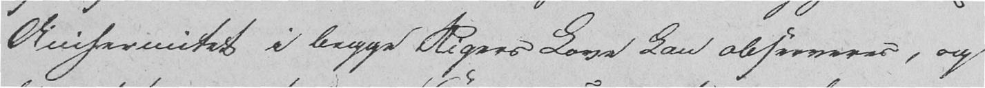Aniseenitet i begge Rigers Love kan observeres, og
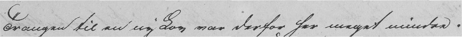Trangen til en ny Lov var derfor her meget mindre.
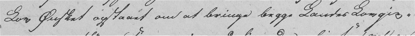Lov Ønsket opstaaet om at bringe begge Landes Lovgiv-
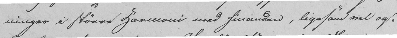ninger i større Harmoni med hinanden, ligesom vel og-
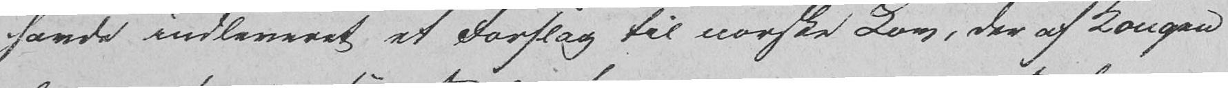havde indleveret et Forslag til norske Lov, der af Kongen
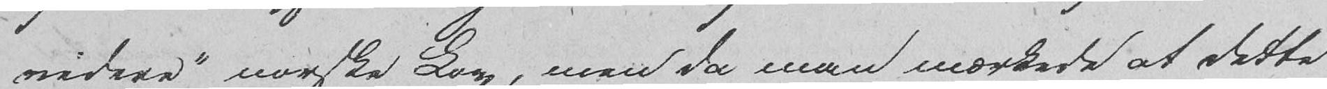videre" norske Lov, men da man mærkede at dette
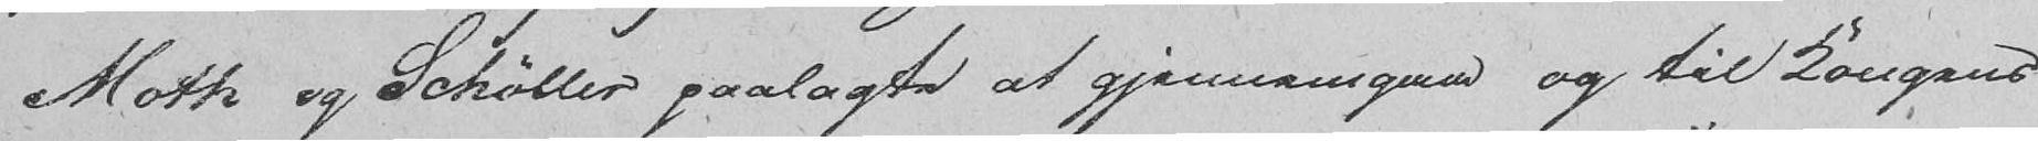Moth og Schøller paalagte at gjennemgaa og til Kongens
